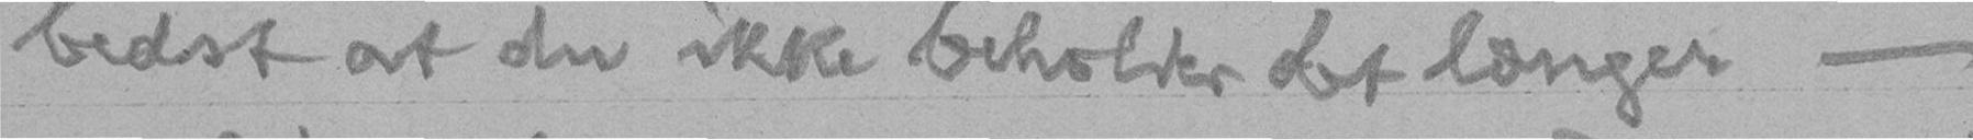bedst at du ikke beholder det længer -
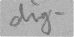dig.
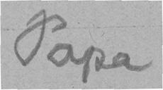Papa
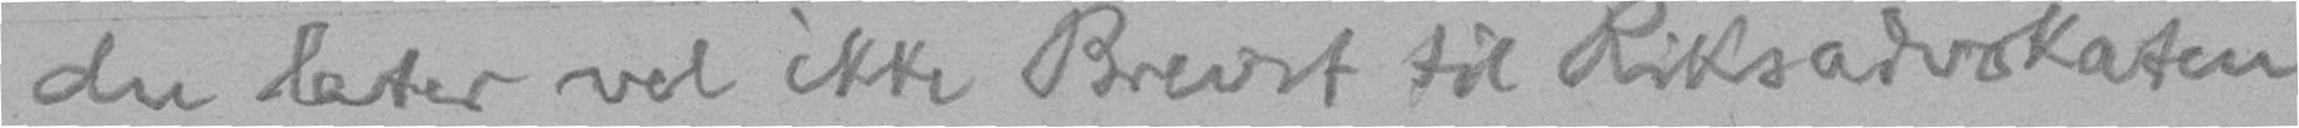du later vel ikke Brevet til Riksadvokaten
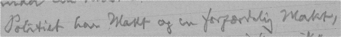Politiet har Makt og en forfærdelig Makt,
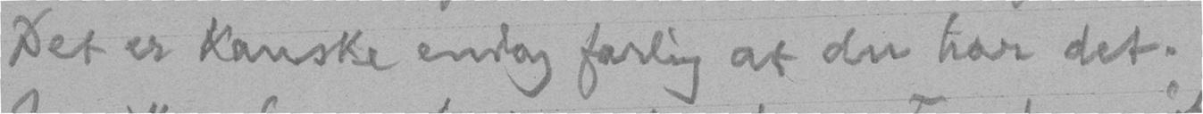Det er kanske endog farlig at du har det.
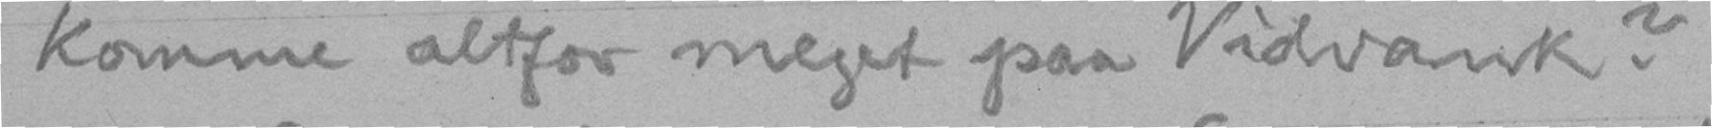komme altfor meget paa Vidvank?
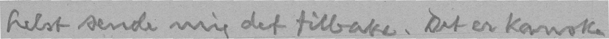helst sende mig det tilbake. Det er kanske
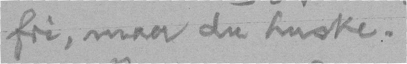fri, maa du huske.
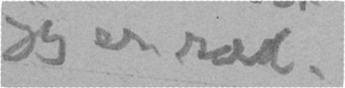jeg er ræd.
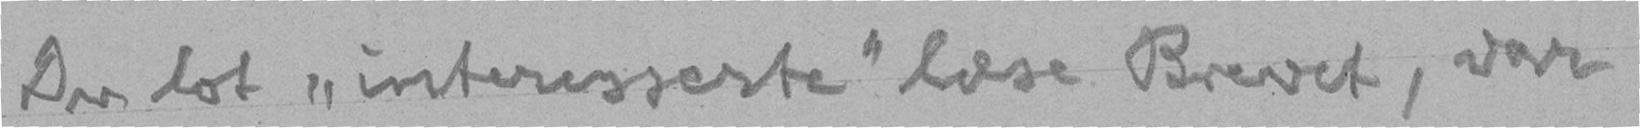Du lot "interesserte" læse Brevet, var
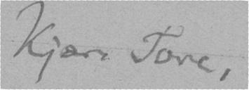Kjære Tore,
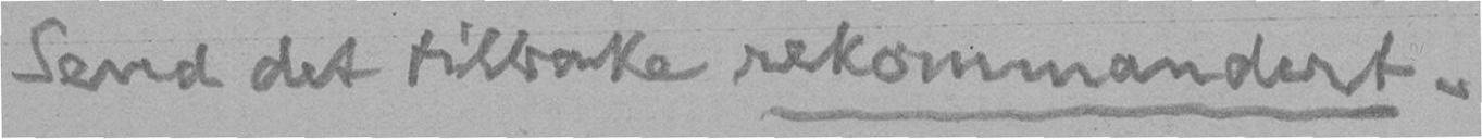Send det tilbake rekommandert.
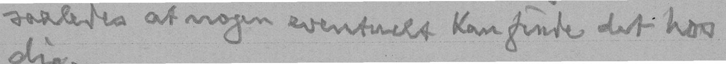saaledes at nogen eventuelt kan finde det hos
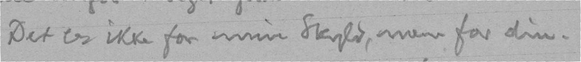Det er ikke for min Skyld, men for din.
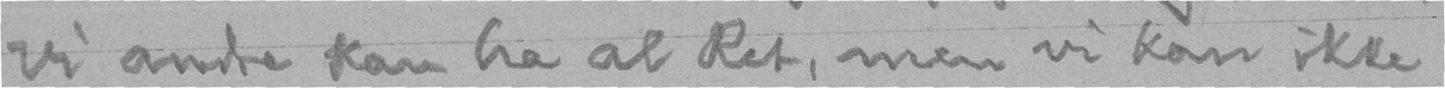vi andre kan ha al Ret, men vi kan ikke
